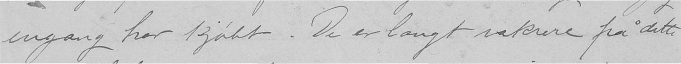engang har kjøbt. De er langt vakrere på dette
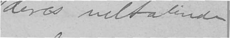deres veltalende
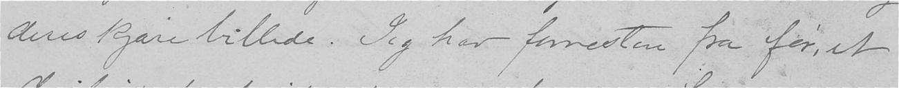deres kjære billede. Jeg har forresten fra før, et
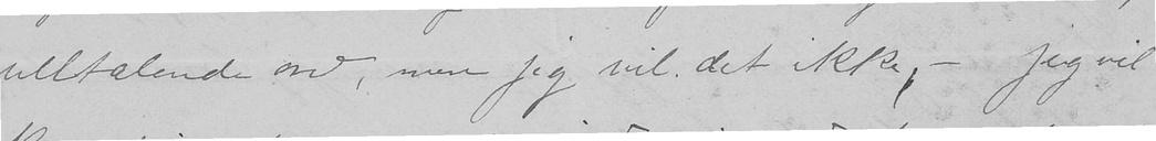veltalende ord, men jeg vil det ikke, - jeg vil
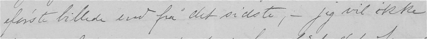første billede end på det sidste, - jeg vil ikke
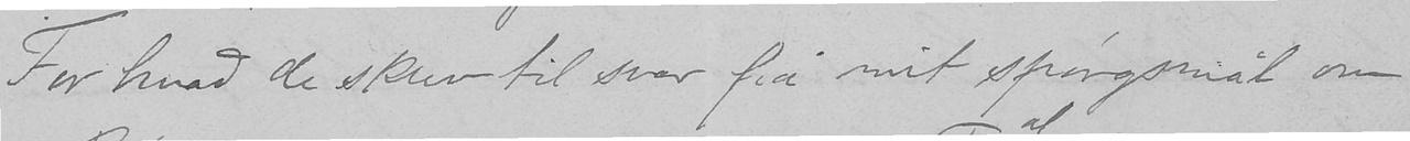For hvad de skrev til svar på mit spørgsmål om
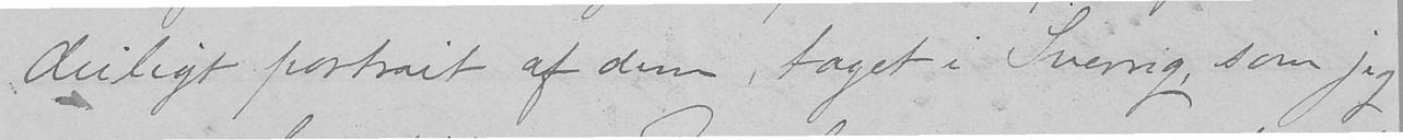deiligt portrait af dem, taget i Sverrig, som jeg
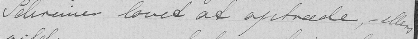Schreiner lovet at optræde, - ellers
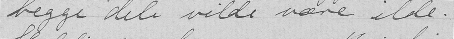begge dele vilde være ilde.
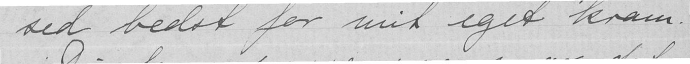sed bedst for mit eget kram.
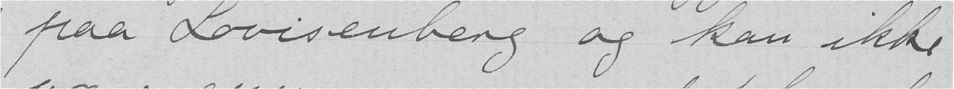paa Lovisenberg og kan ikke
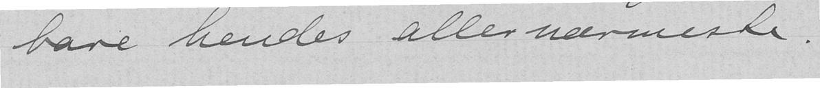bare hendes aller nærmeste.
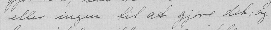eller ingen til at gjøre det; og
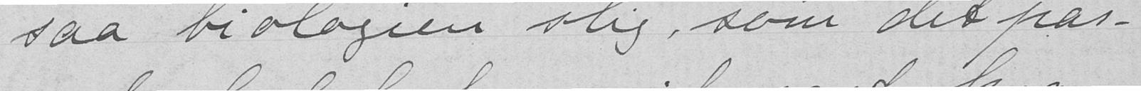saa biologien slig, som det pas-
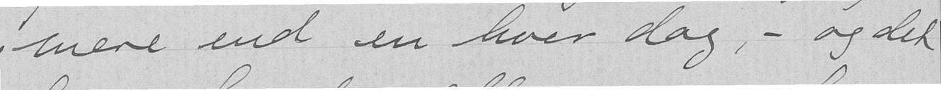mere end en hver dag, - og det
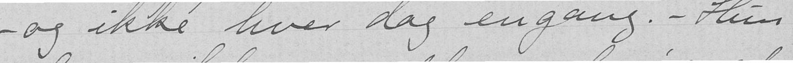- og ikke hver dag engang. - Hun
